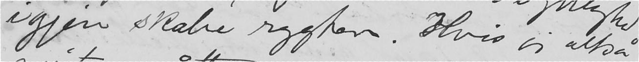igjen skabe rygter. Hvis jeg altså
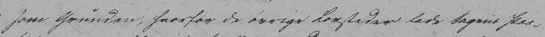som Grunden, hvorfor de øvrige Lovsteder lade Sagens Paa-
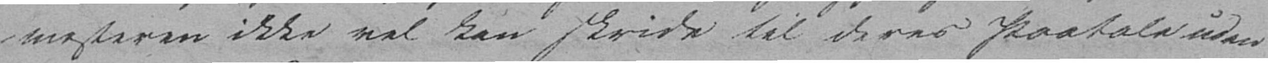mesteren ikke vel kan skride til deres Paatale uden
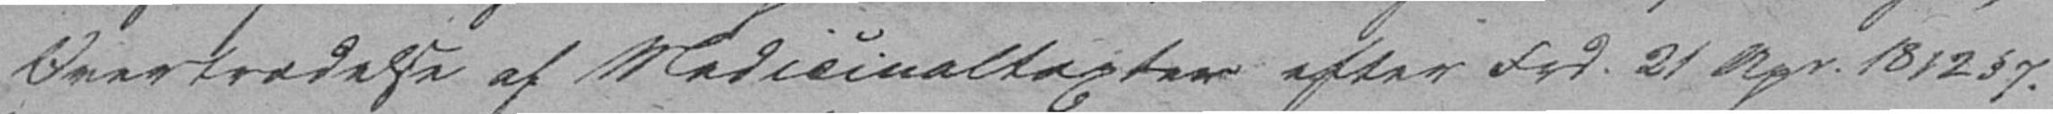Overtrædelse af Medicinaltaxter efter Frd. 21 Apr. 1812 § 7.
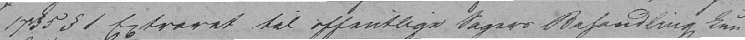1735 § 1 Extraret til offentlige Sagers Behandling, kun
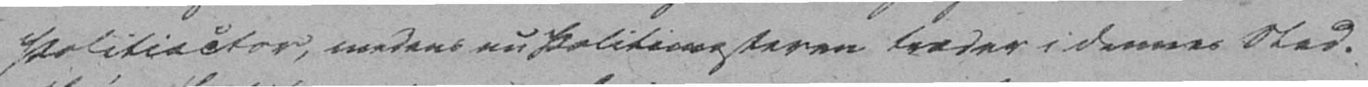Politiactor, medens nu Politimesteren træder i dennes Sted.
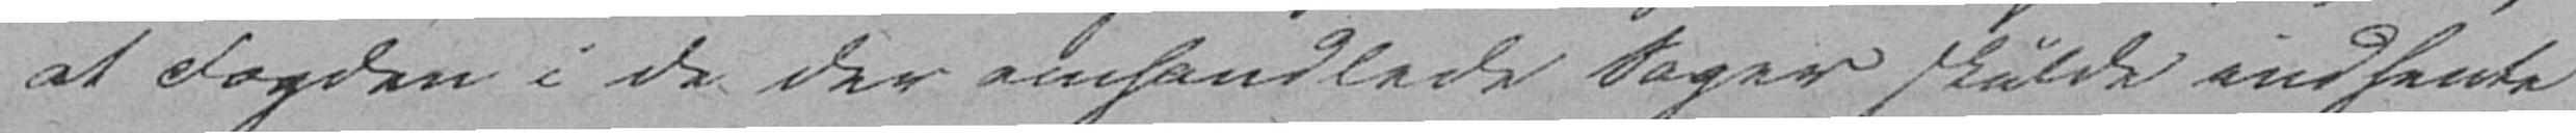at Fogden i de der omhandlede Sager skulde indhente
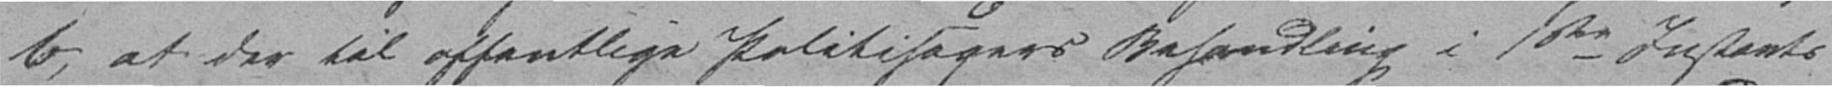b, at der til offentlige Politisagers Behandling i 1ste Instants
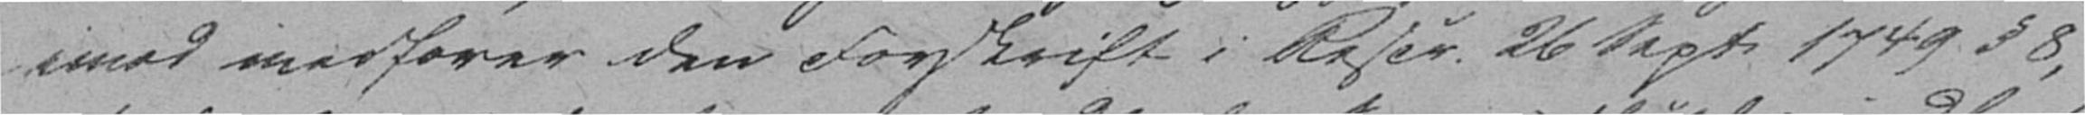imod medfører den Forskrift i Rescr. 26 Sept 1749 § 8,
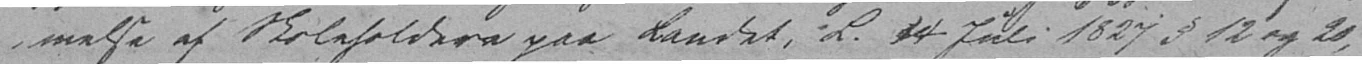melse af Skoleholdere paa Landet, L. 14 Juli 1827 § 12 og 20,
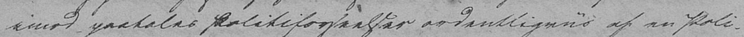imod paatales Politiforseelser ordentligviis af en Poli-
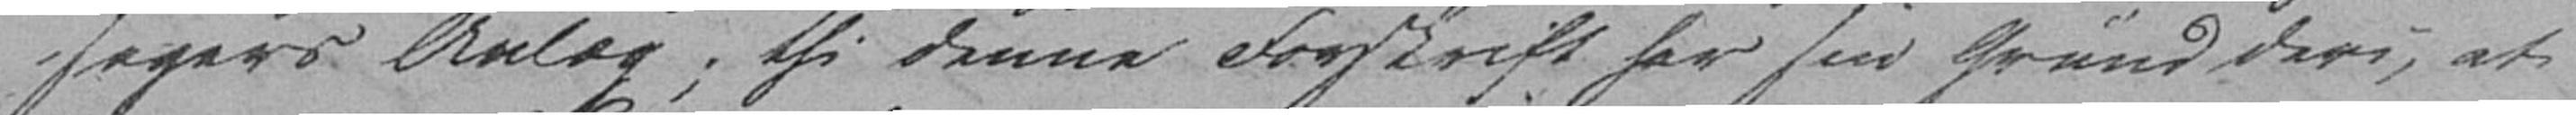sagers Anlæg; thi denne Forskrift har sin Grund deri, at
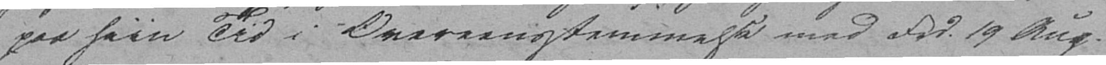paa hiin Tid i Overeensstemmelse med Frd. 19 Aug.
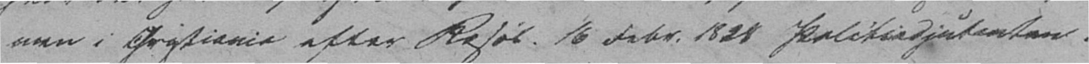men i Christiania efter Rescr. 16 Febr. 1828 Politiadjutanten.
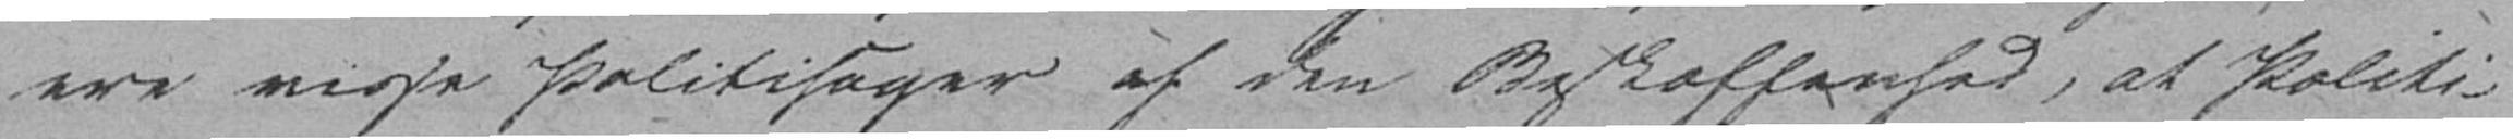ere visse Politisager af den Beskaffenhed, at Politi-
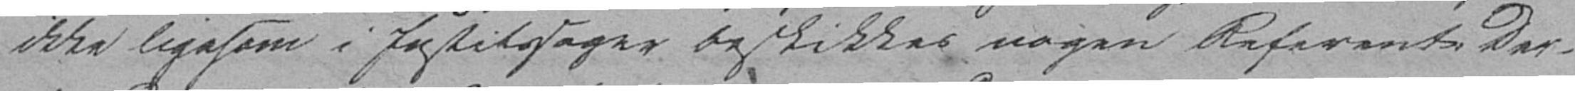ikke ligesom i Justitssager beskikker nogen Referent. Der-
